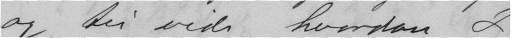og til vide, hvordan I
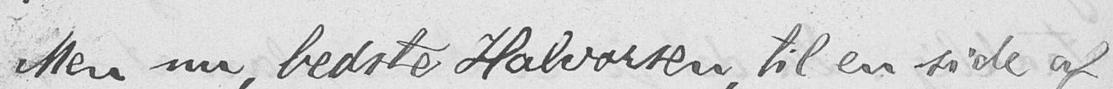Men nu, bedste Halvorsen, til en side af
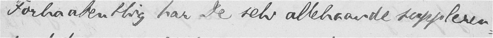Forhaabentlig har De selv allehaande suppleren-
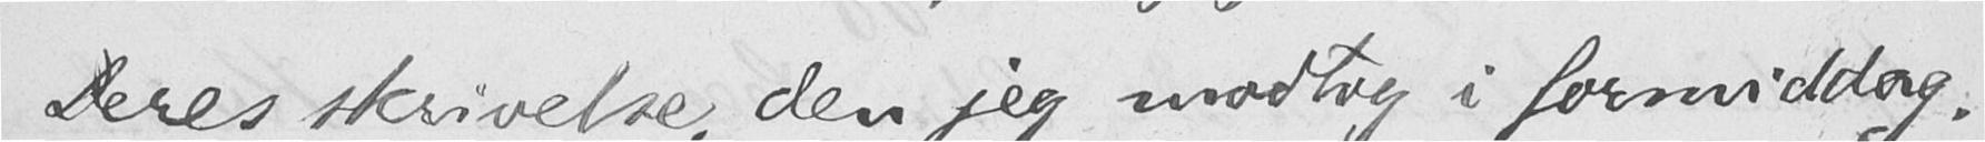Deres skrivelse, den jeg modtog i formiddag.
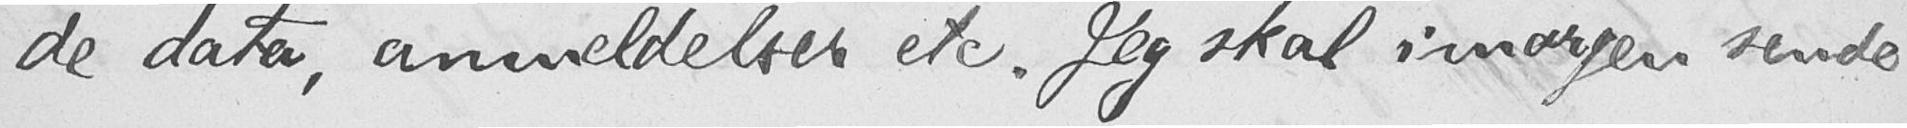de data, anmeldelser etc. Jeg skal imorgen sende
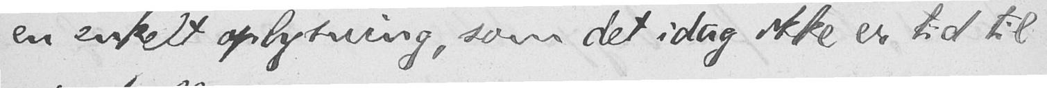en enkelt oplysning, som det idag ikke er tid til
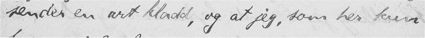sender en art kladd, og at jeg, som her kun
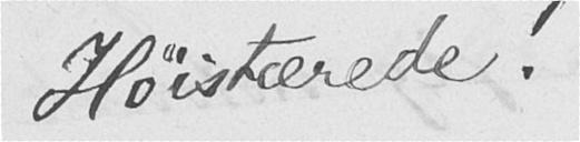Høistærede!
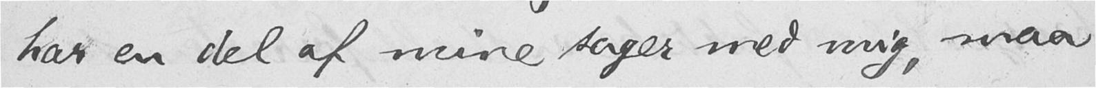har en del af mine sager med mig, maa
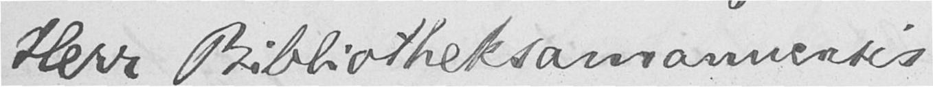Herr Bibliotheksamanuensis
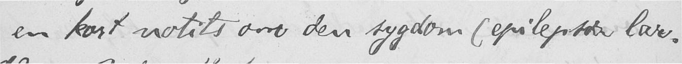en kort notits om den sygdom (epilepsi lar-
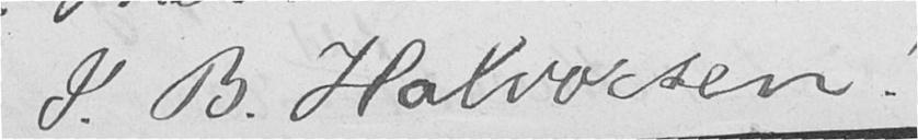J. B. Halvorsen!
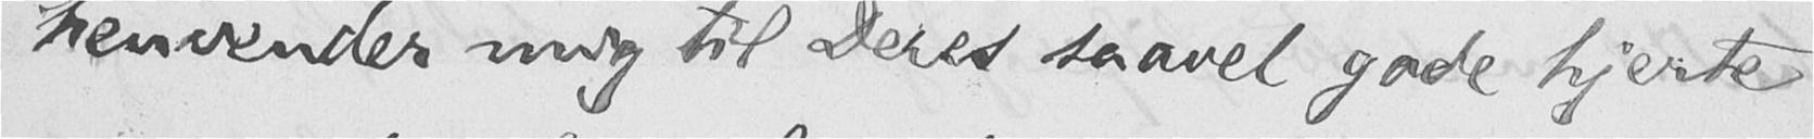henvender mig til Deres saavel gode hjerte
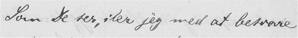Som De ser, iler jeg med at besvare
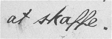at skaffe.
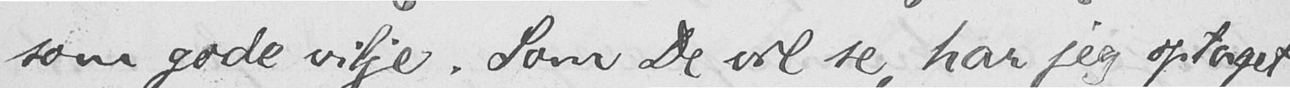som gode vilje. Som De vil se, har jeg optaget
